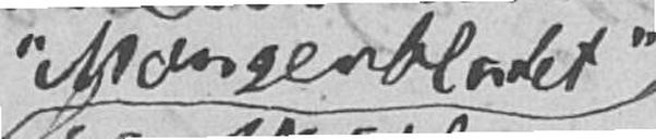"Morgenbladet"
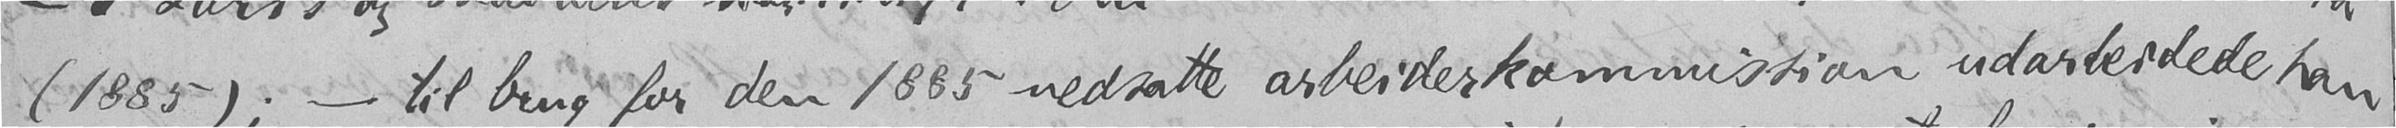(1885); - til brug for den 1885 nedsatte arbeiderkommission udarbeidede han
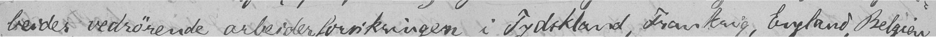beider, vedrørende arbeiderforsikringen, i Tydskland, Frankrig, England, Belgien,
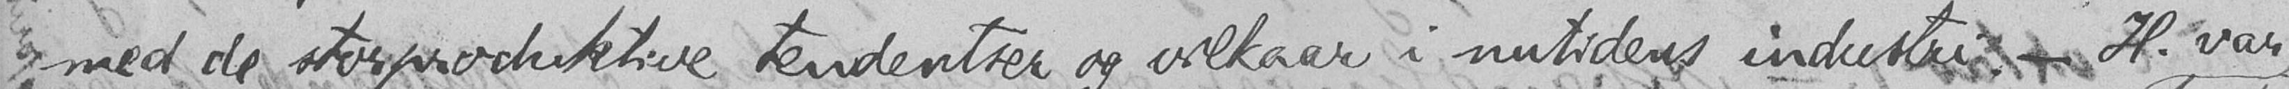med de storproduktive tendentser og vilkaar i nutidens industri. - H. var
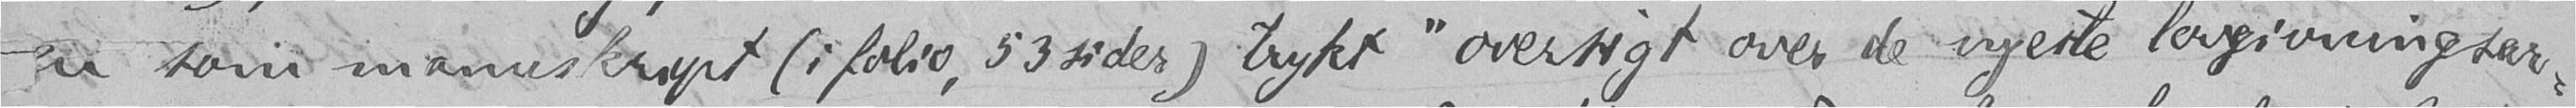en som manuskript (i folio, 53 sider) trykt "oversigt over de nyeste lovgivningsar-
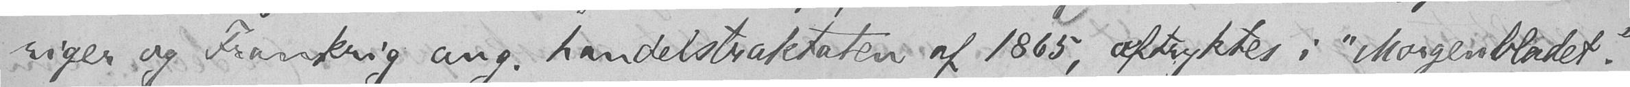riger og Frankrig ang. handelstraktaten af 1865, aftryktes i "Morgenbladet".
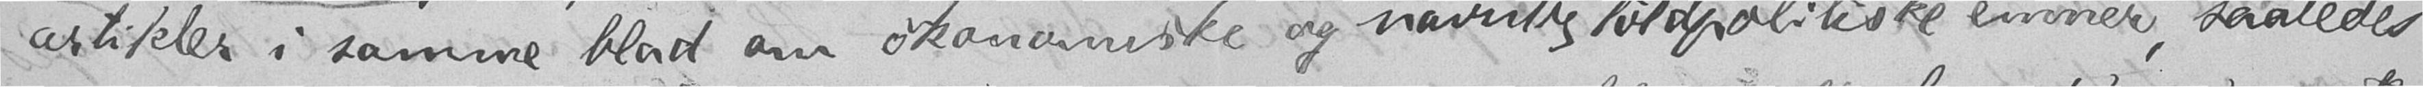artikler i samme blad om økonomiske og navnlig toldpolitiske emner, saaledes
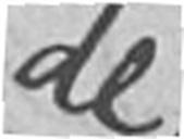de
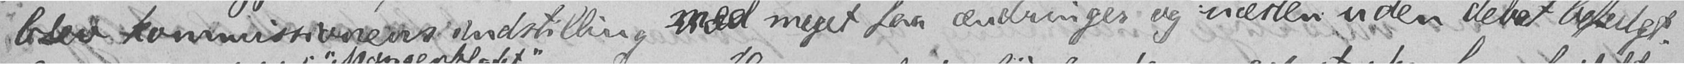blev kommissionens indstilling med meget faa ændringer og næsten uden debat befulgt.
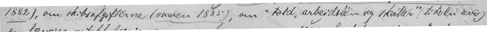1882), om skibsafgifterne (vaaren 1885), om "told, arbeidsløn og skatter" (titelen uvis)
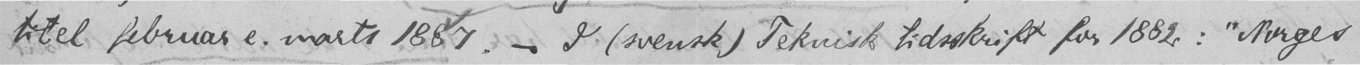titel februar e. marts 1887. - I (svensk) Teknisk tidsskrift for 1882: "Norges
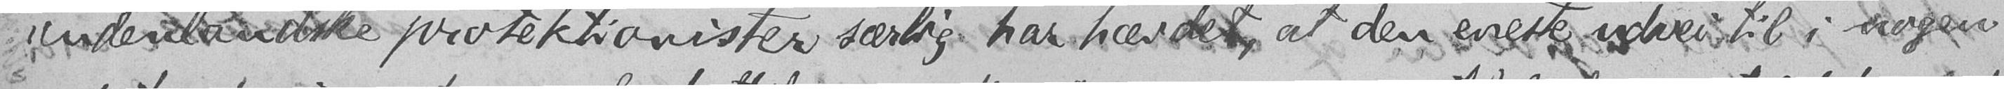udenlandske protektionister særlig har hævdet, at den eneste udvei til i nogen
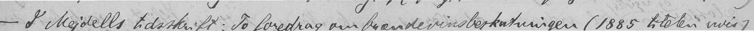- I Mejdells tidsskrift: To foredrag om brændevinsbeskatningen (1885, titelen uvis).
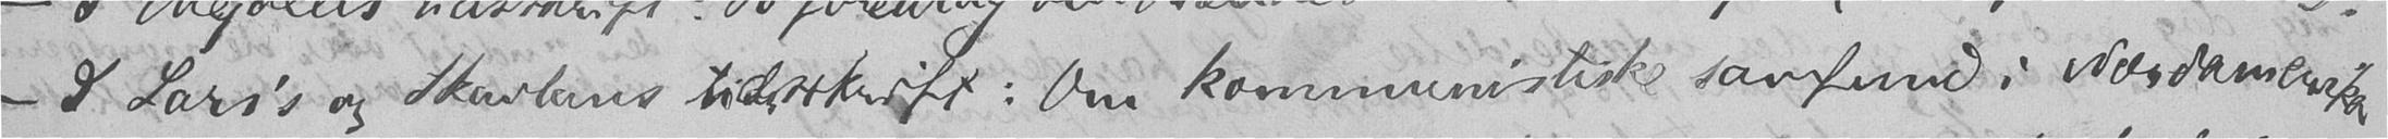- I Lars's og Skavlans tidsskrift: Om kommunistiske samfund i Nordamerika
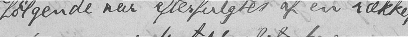følgende aar efterfulgtes af en række
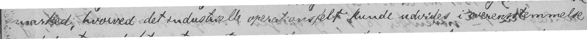marked, hvorved det industrielle operationsfelt kunde udvides i overensstemmelse
